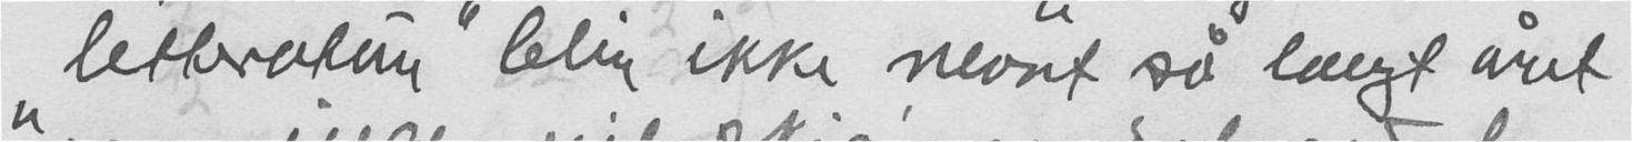"litteratur" blir ikke nevnt så langt året
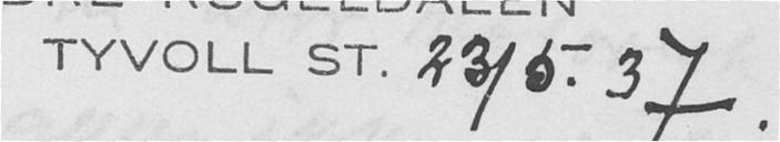TYVOLL ST. 23/5. 37.
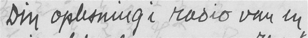Din oplesning i radio var en
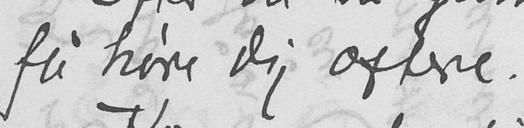få høre Dig oftere.
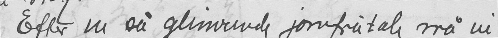Efter en så glimrinde jomfrutale må vi
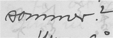sommer?
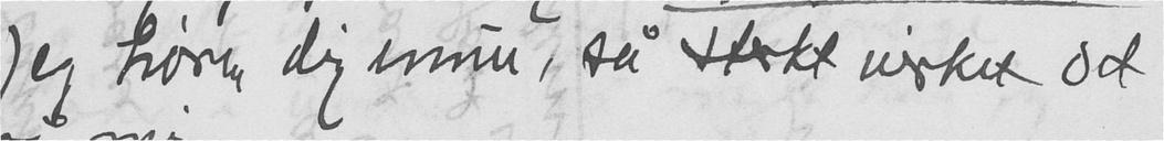Jeg hører dig ennu, så sterkt virket det
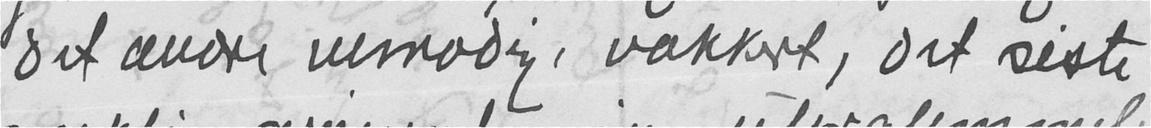det andre vemodig, vakkert, det siste
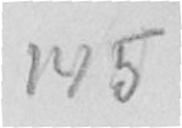145
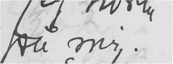på mig.
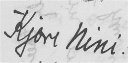Kjære Nini.
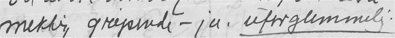mektig gripende - ja, uforglemmelig.
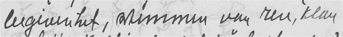begivenhet, stemmen var ren, klar
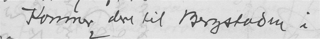Kommer dere til Bergstaden i
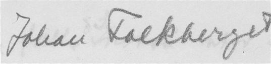Johan Falkeberget
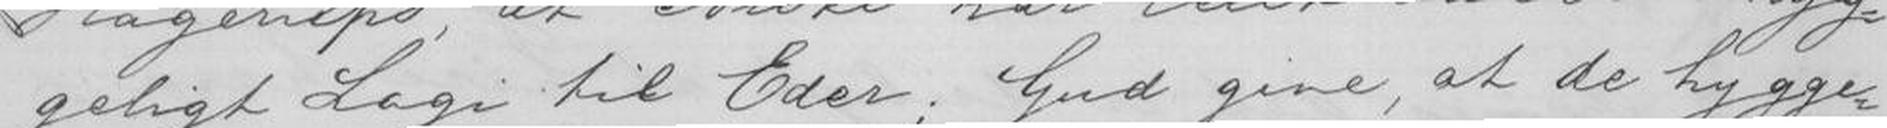geligt Logi til Eder; Gud give, at de hygge-
